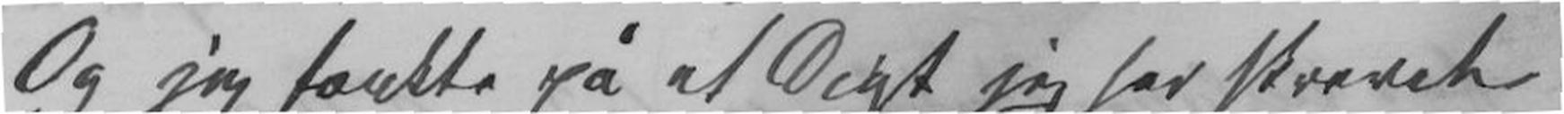Og jeg tænkte på et Digt jeg har skrevet
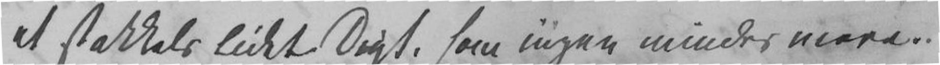et stakkels lidet Digt, som ingen mindes mere.
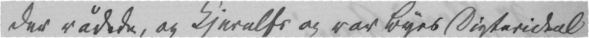der rådede, og Kjerulfs og var Byes Digterideal
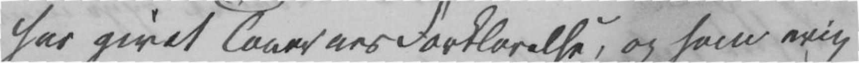har givet Toner ens Forklarelse, og som mig
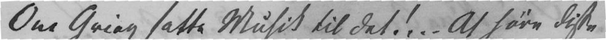Om Grieg satte Musik til det!.. At høre Digte
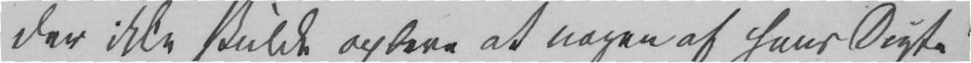der ikke skulde opleve at nogen af hans Digte blevv
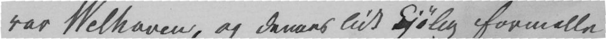var Welhaven, og damers lidt Kjølig formelle
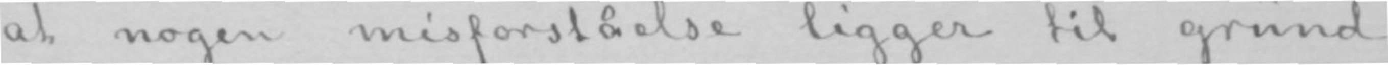at nogen misforståelse ligger til grund
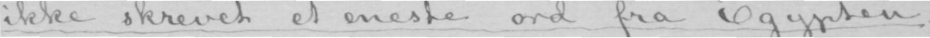ikke skrevet et eneste ord fra Egypten.
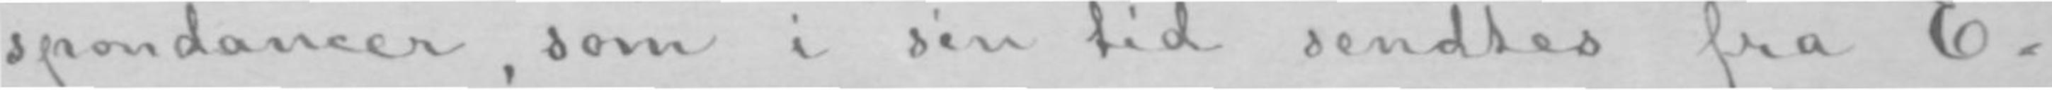spondancer, som i sin tid sendtes fra E-
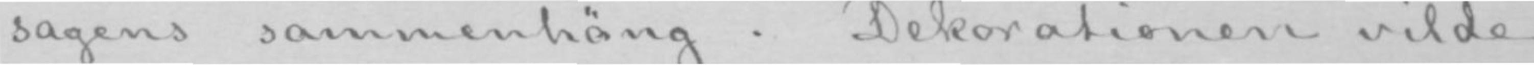sagens sammenhäng. Dekorationen vilde
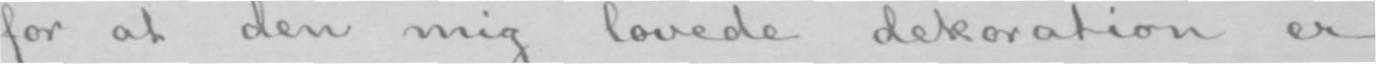for at den mig lovede dekoration er
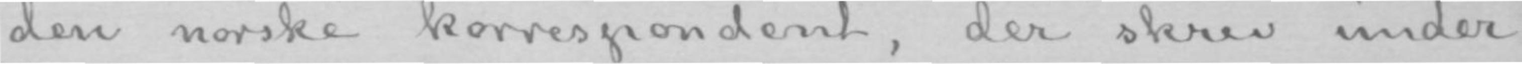den norske korrespondent, der skrev under
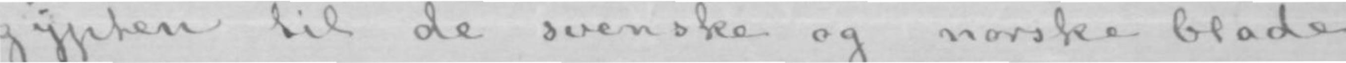gypten til de svenske og norske blade.
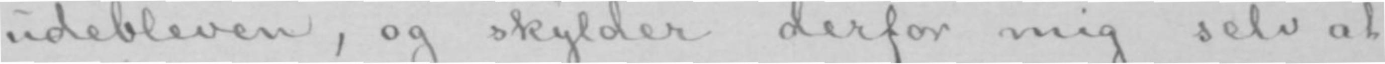udebleven, og skylder derfor mig selv at
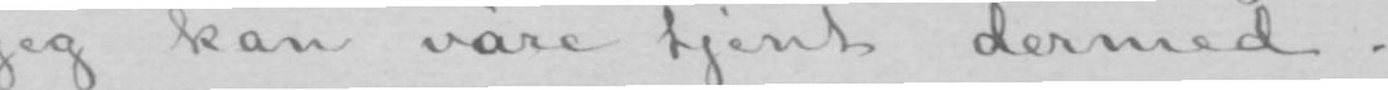jeg kan väre tjent dermed.
